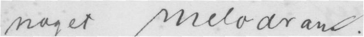noget Melodrama
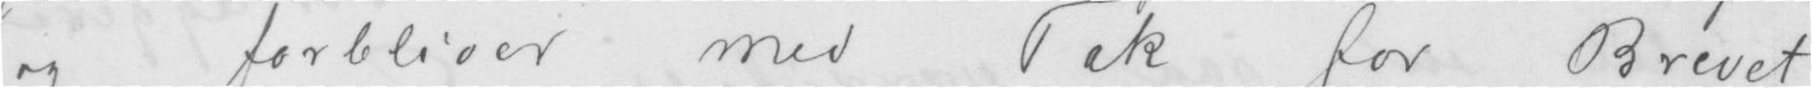og forbliver med Tak for Brevet
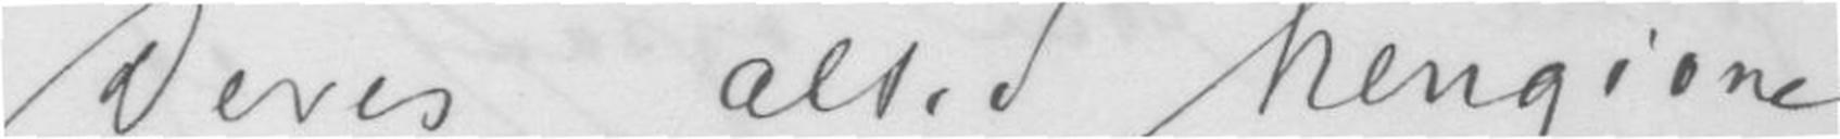Deres altid Hengivne
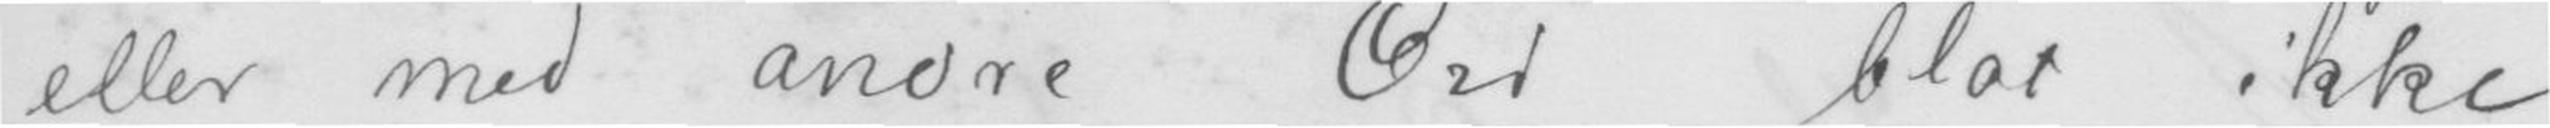eller med andre Ord blot ikke
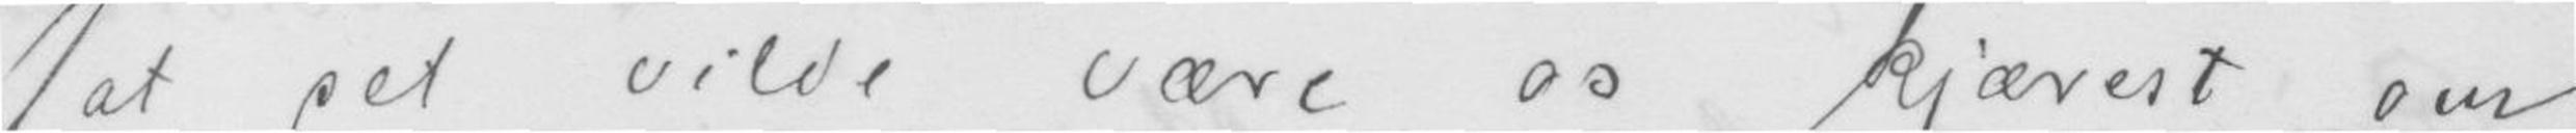at det vilde være os kjærest om
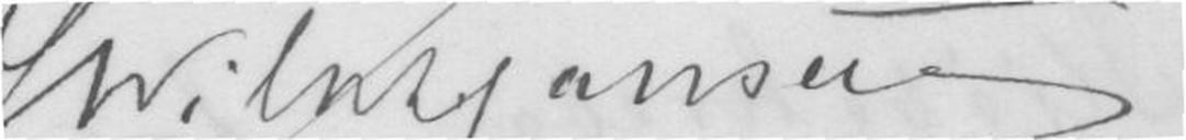Wilhelm Hansen
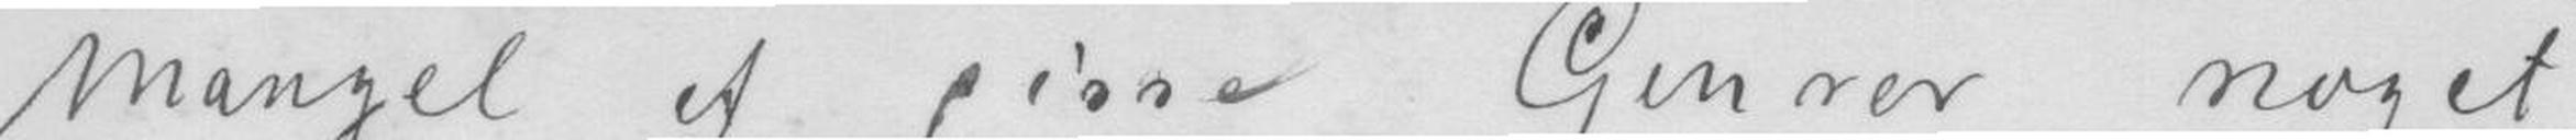mangel af disse Genrer noget
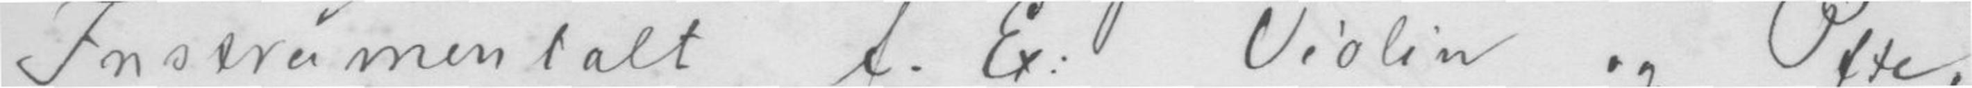Instrumentalt f.Ex. Violin og Ofte,
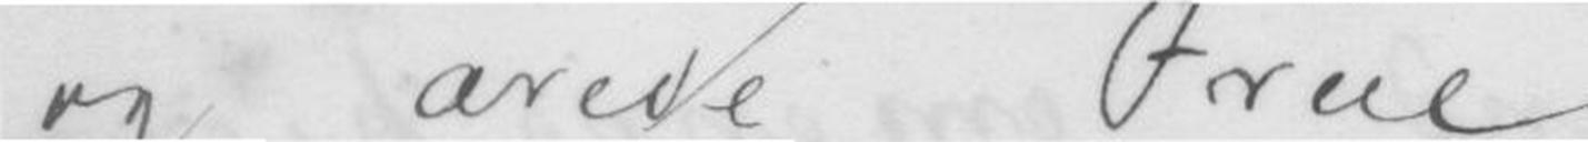og ærede Frue.
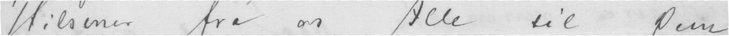Hilsener fra os Alle til Dem
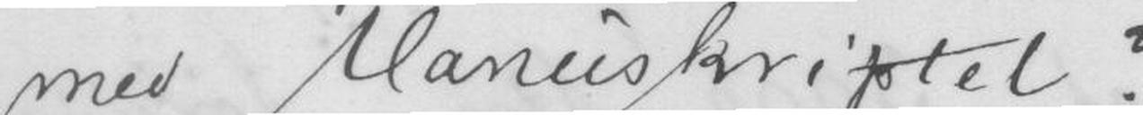med Manuskriptet?
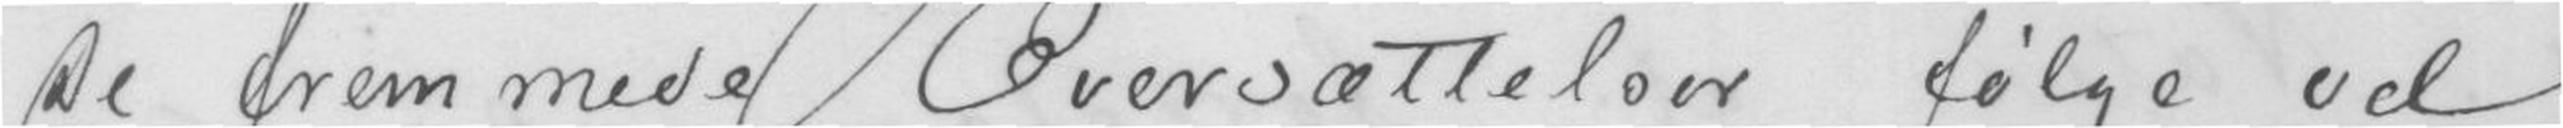De fremmede Oversættelser følger ud
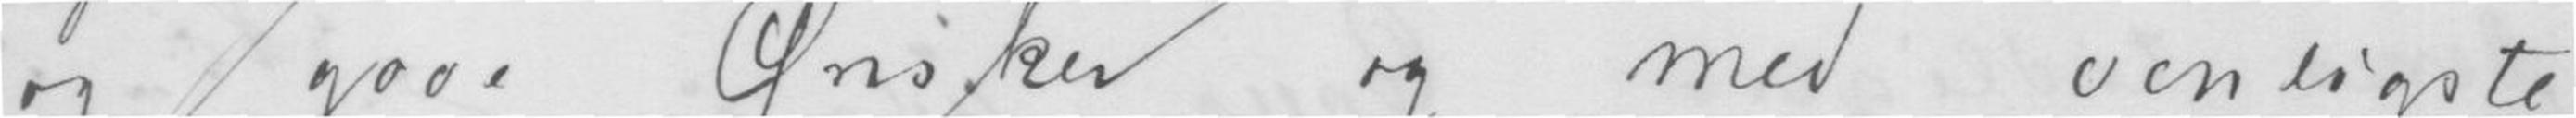og gode Ønsker og med venligste
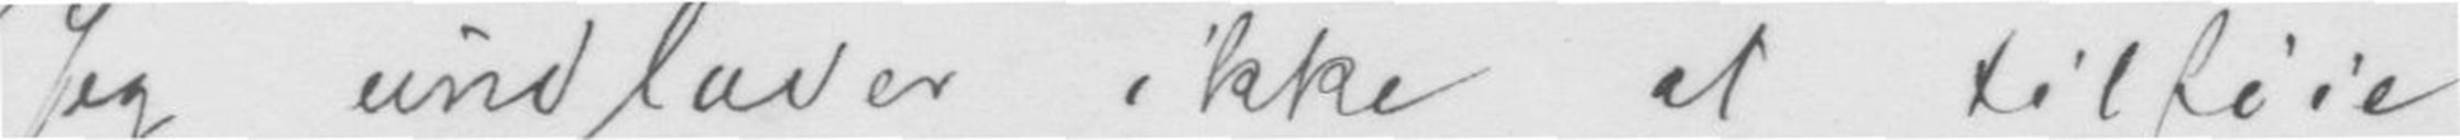Jeg undlader ikke at tilføie,
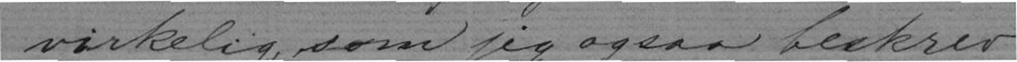virkelig, som jeg ogsaa beskrev
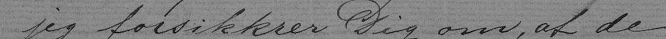jeg forsikkrer Dig om, at de
